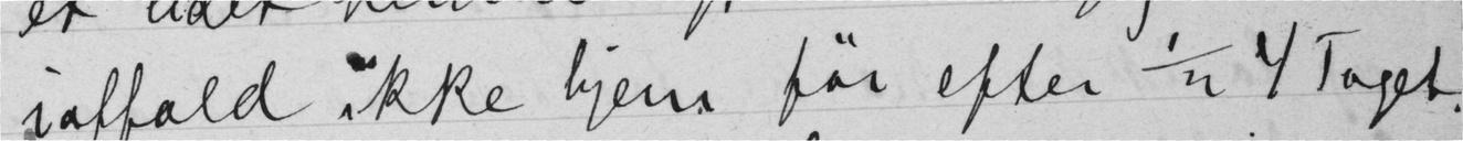iaffald ikke hjem før efter ¼ 4 Toget.
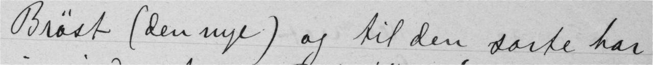Brøst (den nye) og til den sorte har
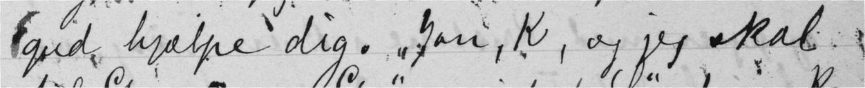gud hjælpe dig. Jan, K, og jeg skal
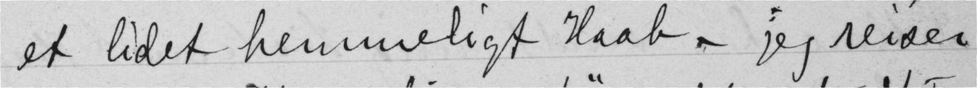et lidet hemmeligt Haab.- jeg reiser
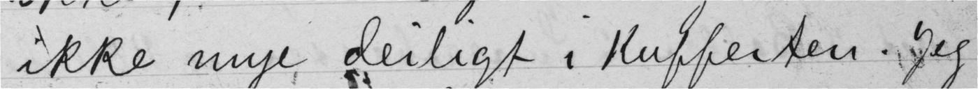ikke mye deiligt i Kufferten. Jeg
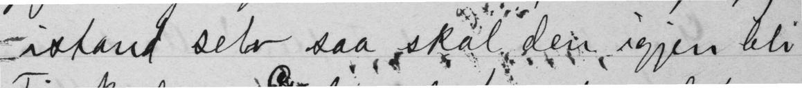istand selv saa skal den igjen bli
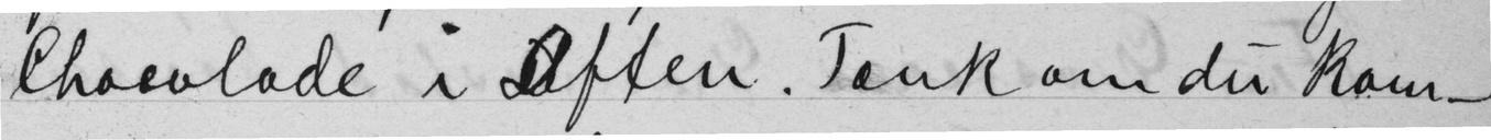Chocolade i Aften. Tænk om du Kom -
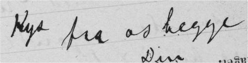Kys fra os begge
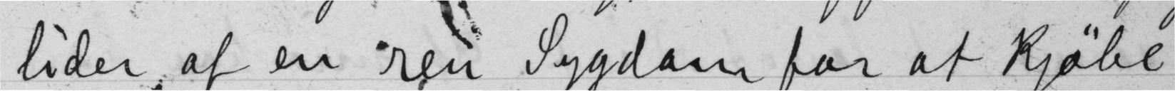lider af en ren sygdom for at Kjøbe
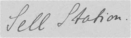Sell Station.
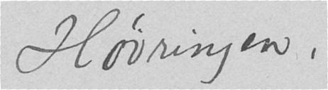Høvringen.
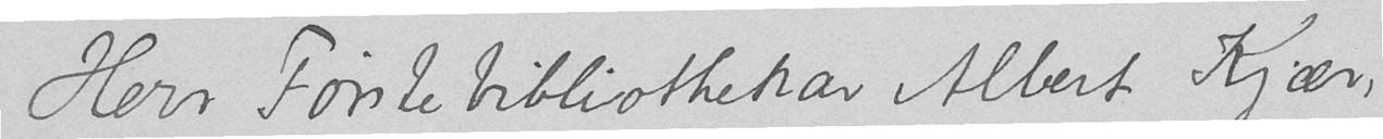Herr Førstebibliothekar Albert Kjær,
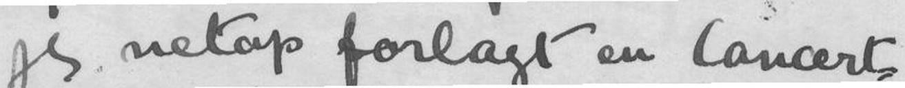jeg netop forlagt en Concert-
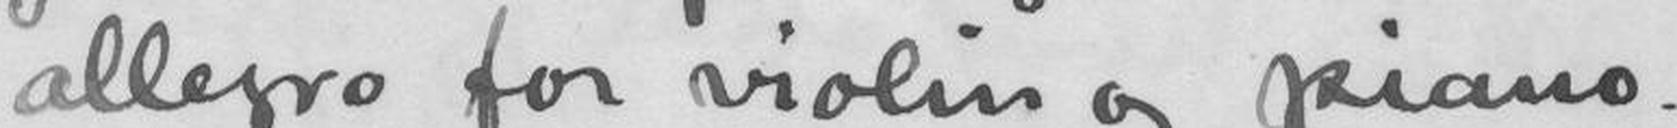allegro for violin og piano.
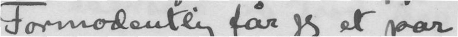Formodentlig får jeg et par
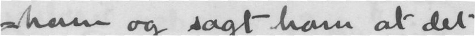ham og sagt ham at det
